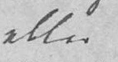eller
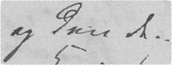og den der-
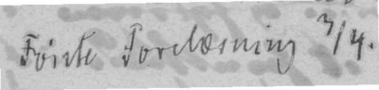Første Forelæsning 3/4
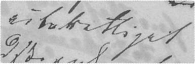uberettiget
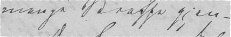mange Straffe gjen-
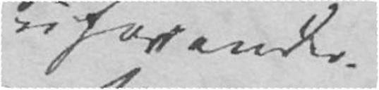uforander-
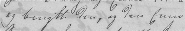og benytte den, og den Evne
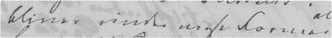bliver under visse Former
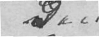den
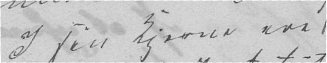I sin Kjerne ere
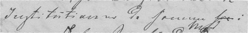Institutioner de samme i
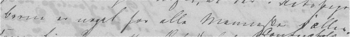berne er noget for alle Mennesker fælles.
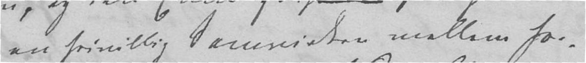en frivillig Samvirken mellem for-
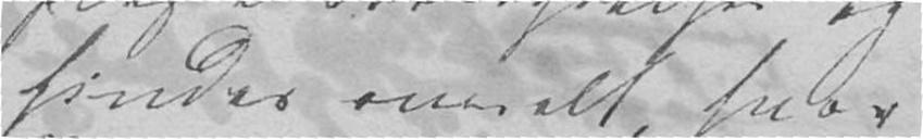findes overalt hvor
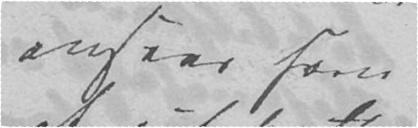ansees som
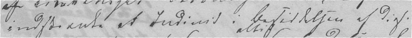indskrænke et Individ i Besiddelsen af disse
